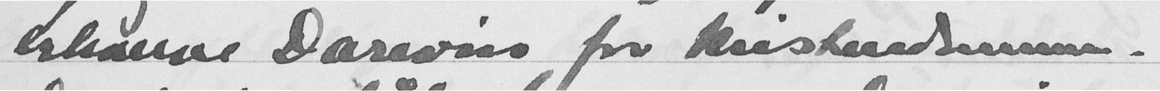lstioerve Darwin for kristendommen.
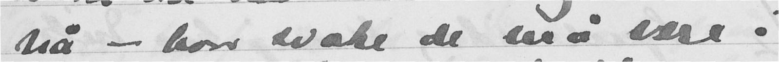nå - hvor svake de må være i
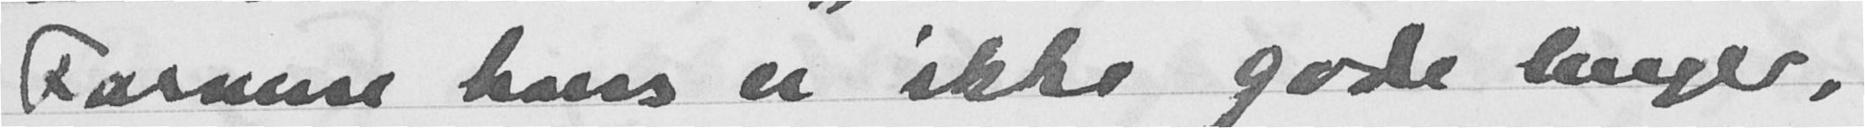Farsene hans er ikke gode lenger,
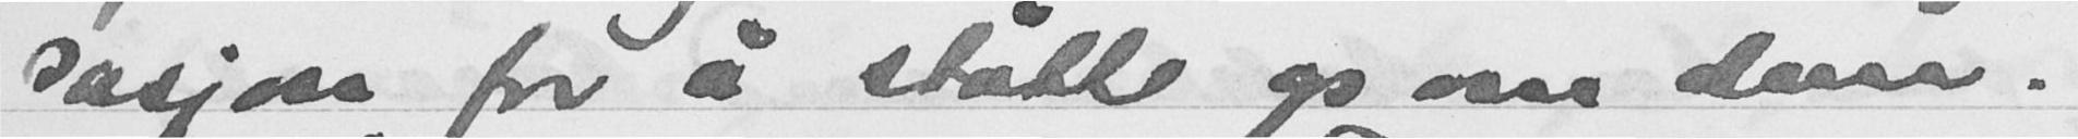sasjon for å støtte op om dem.
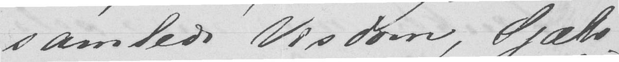samlede Visdom, Sjæls-
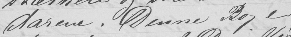Aarene. Denne Bog er
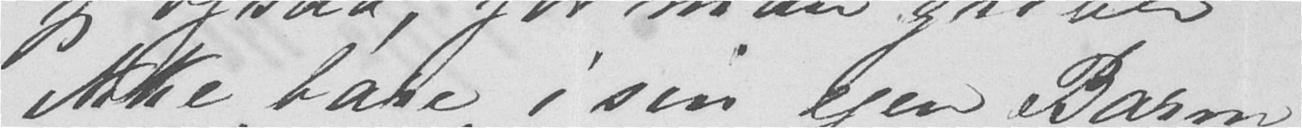ikke bare i sin egen Barm.
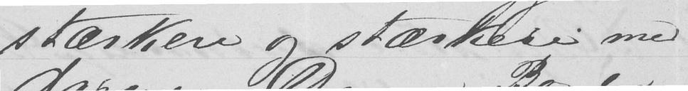stærkere og stærkere med
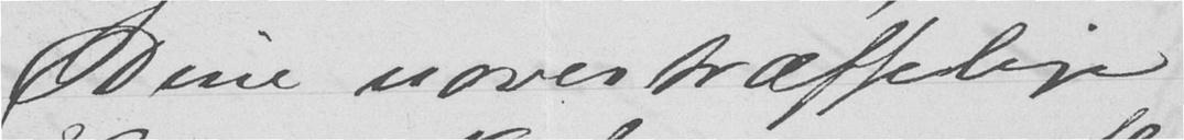Dine uovertræffelige
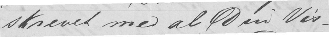skrevet med al Din Vis-
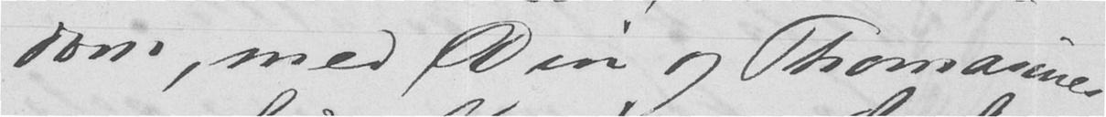dom, med Din og Thomasines
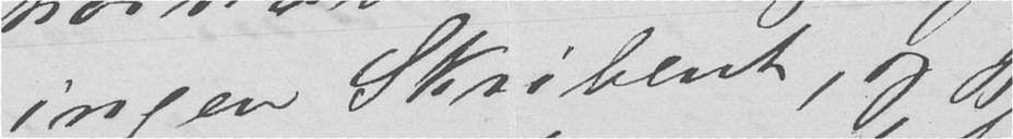ingen Skribent, og jeg
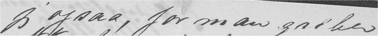jeg ogsaa, for man griber
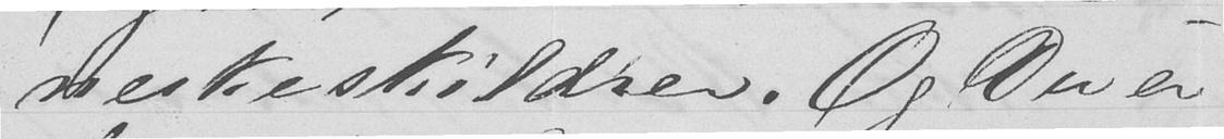neskeskildrer. Og Du er
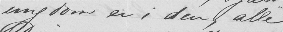ungdom er i den, alle
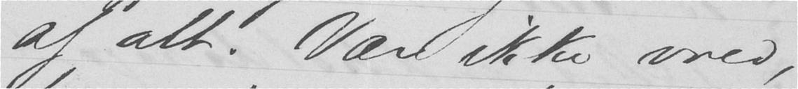af alt! Vær ikke vred,
